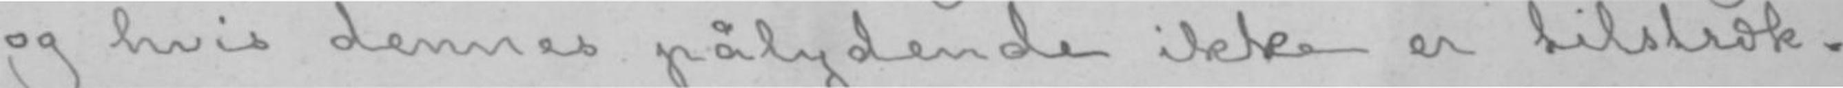og hvis dennes pålydende ikke er tilstræk-
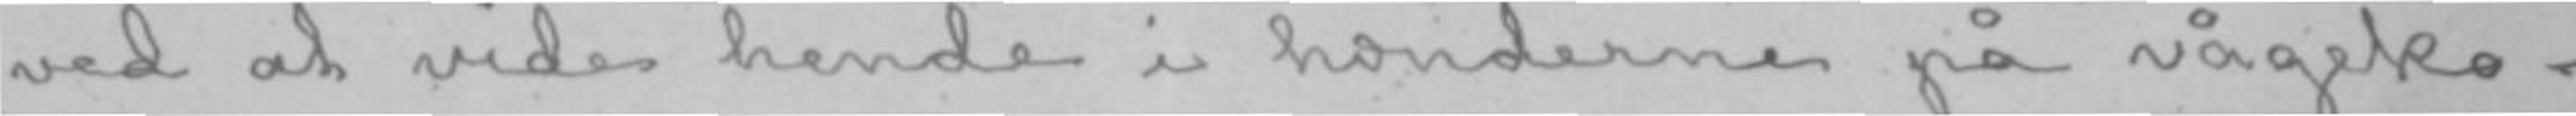ved at vide hende i hænderne på vågeko-
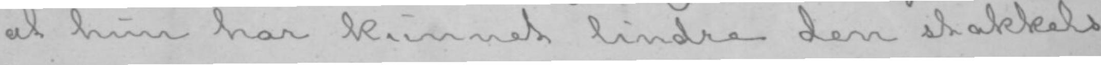at hun har kunnet lindre den stakkels
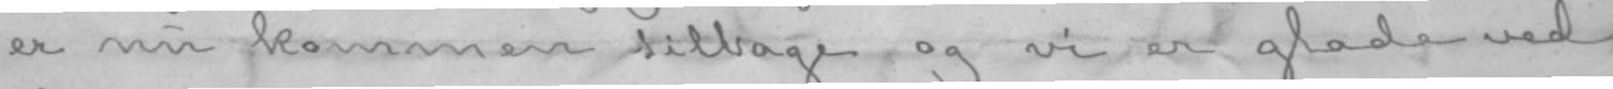er nu kommen tilbage og vi er glade ved
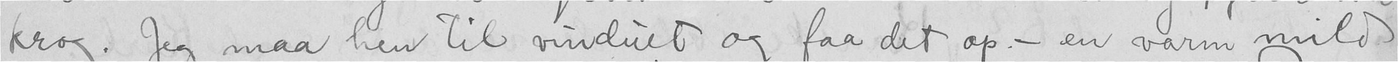krog. Jeg maa hen til vinduet og faa det op - en varm mild
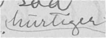hurtiger
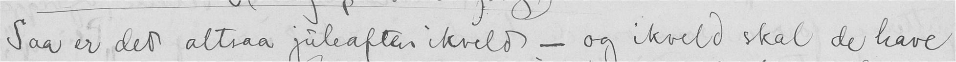Saa er det altsaa juleaften ikveld - og ikveld skal de have
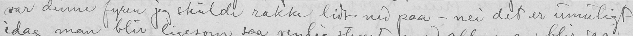var denne fyren jeg skulde rakke lidt ned paa - nei det er umuligt
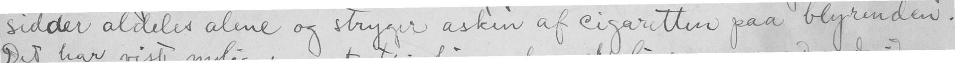sidder aldeles alene og stryger asken af cigaritten paa blyrenden.
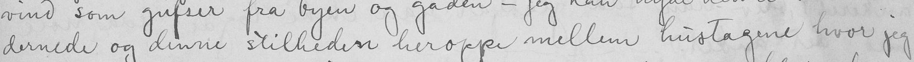dernede og denne stilheden heroppe mellem hustagene hvor jeg
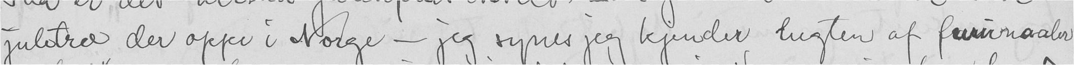julstree der oppe i Norge - jeg synes jeg kjender lugten af furunaaler
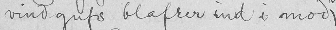vindgufs blafrer ind i mod
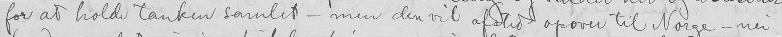for at holde tanken samlet - men den vil afsted opover til Norge - nei
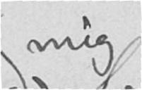mig
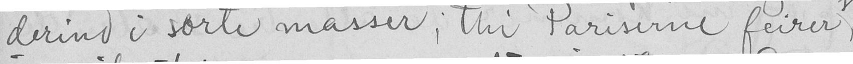derind i sorte masser; thi Pariserne feirer
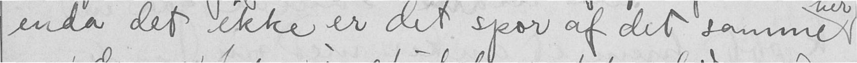enda det ikke er det spor af det samme
